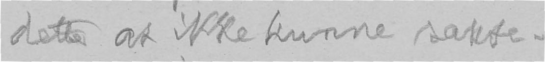dette at ikke kunne sakte.
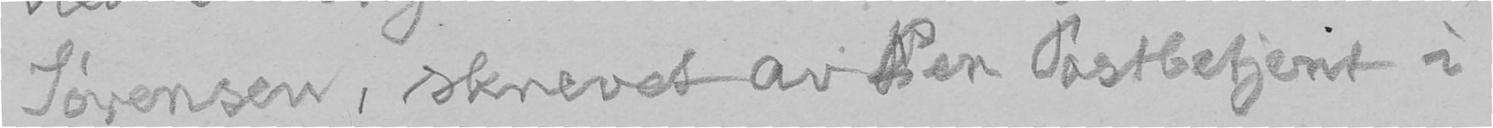Sørensen, skrevet av P en Postbetjent i
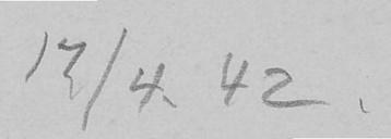17/4. 42.
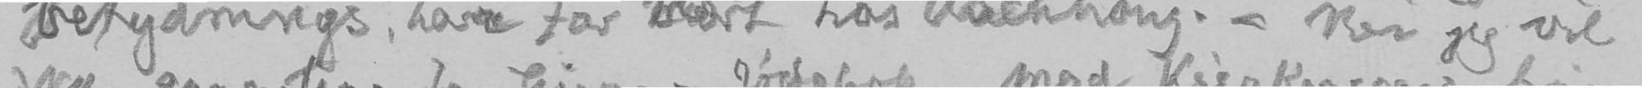betydnings, hare før vært hos Aschehoug. - Nei jeg vil
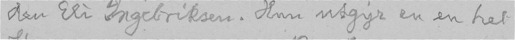den Eli Ingebriksen. Hun utgyr en en hel
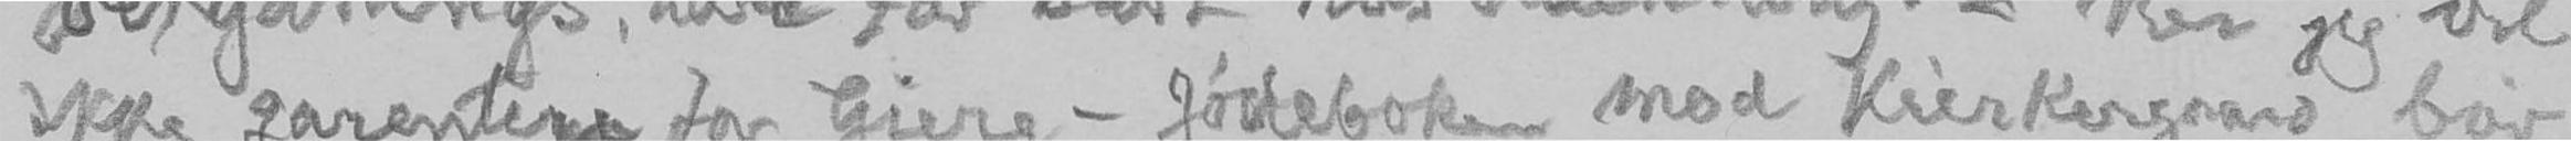ikke garentere for Grieg - Jødeboken mod Kierkegaard bør
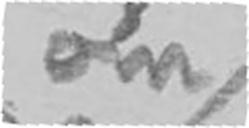om
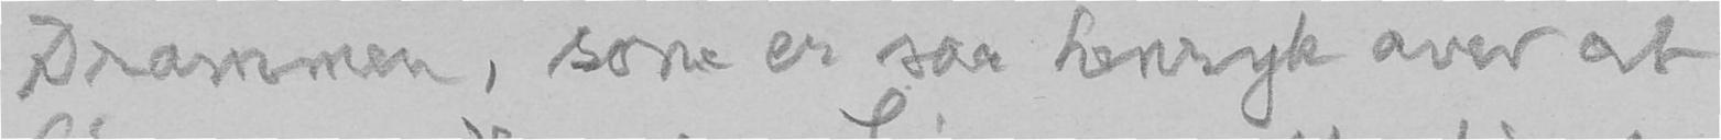Drammen, som er saa henryk over at
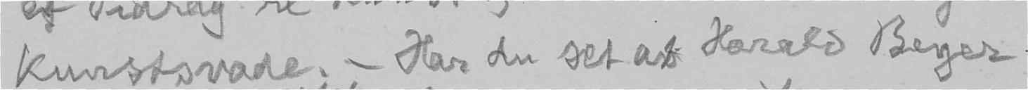kunstsvade. - Har du set at Harald Beyer
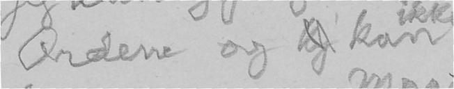Ordene og kj kan
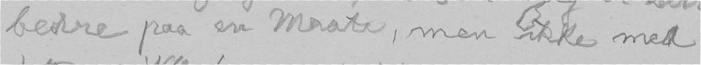bedre paa en Maate, men ikke med
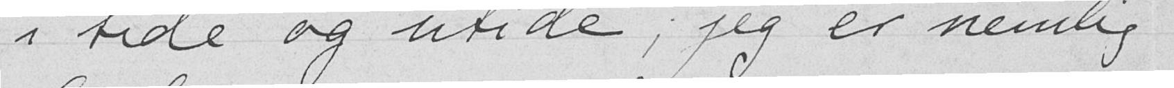i tide og utide, jeg er nemlig
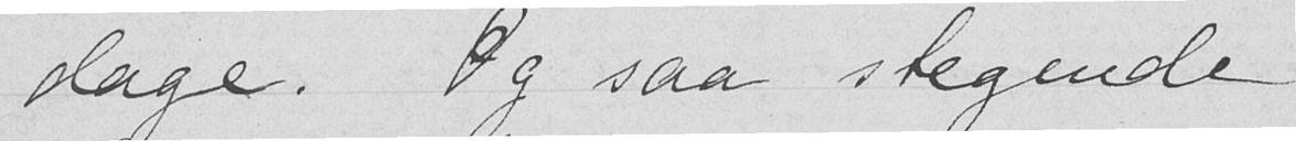dage. Og saa stegende
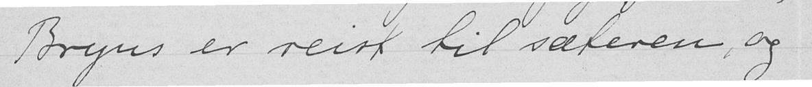Bryns er reist til sæteren, og
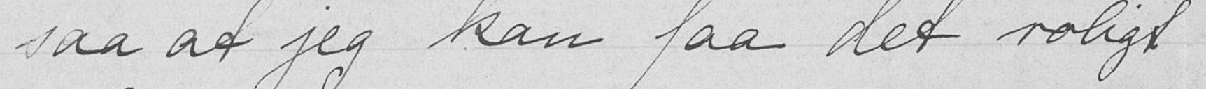saa at jeg kan faa det roligt
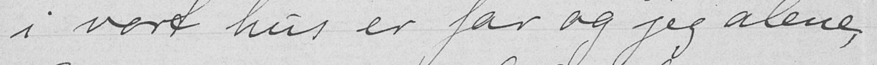i vort hus er far og jeg alene
